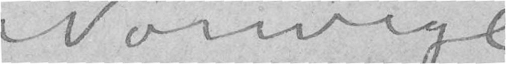Norwege
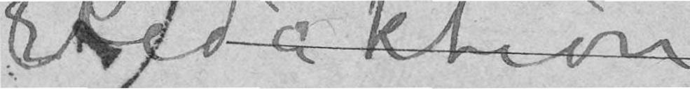Redaktion
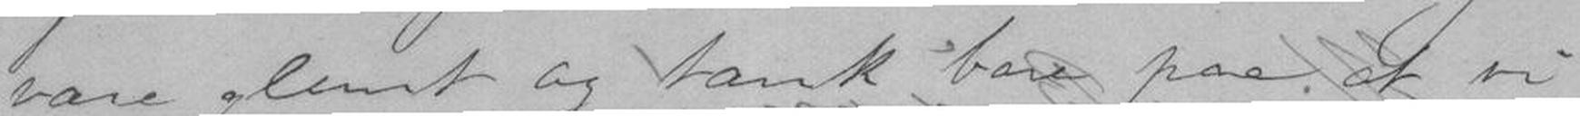være glemt og tænk bare paa at vi
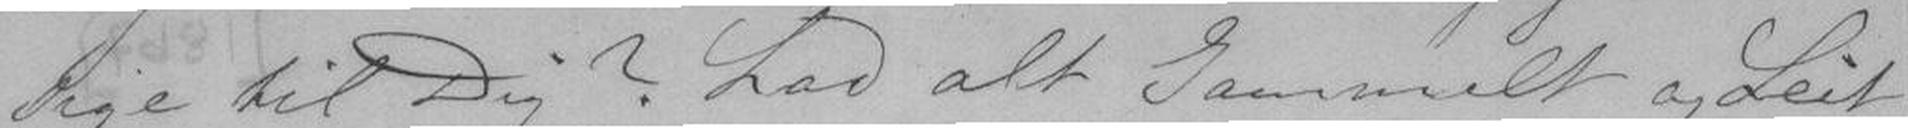sige til Dig? Lad alt Gammelt og Leit
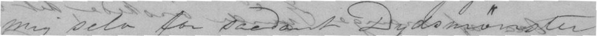mig selv for saadant Dydsmønster
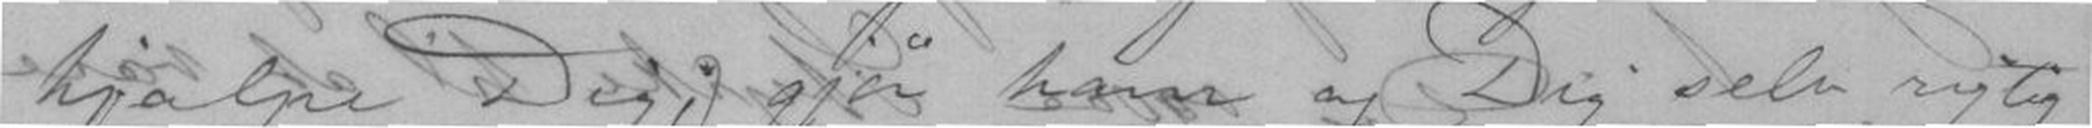hjelpe Dig, gjør ham og Dig selv rigtig
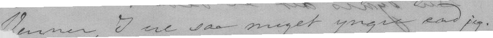Venner, I ere saa meget yngre end jeg.
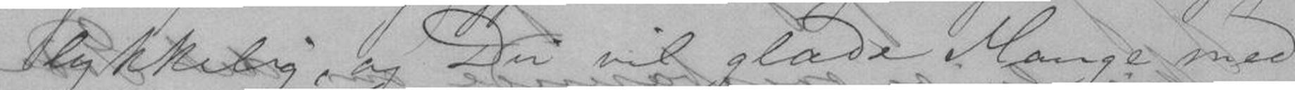lykkelig, og Du vil glæde Mange med
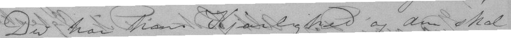Du har hans Kjærlighed og den skal
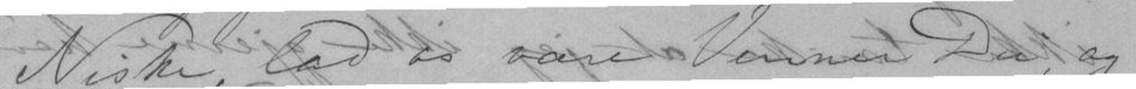Niske, lad os være Venner Du, og
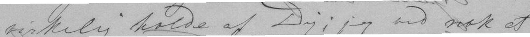virkelig holde af Dig; jeg ved nok at
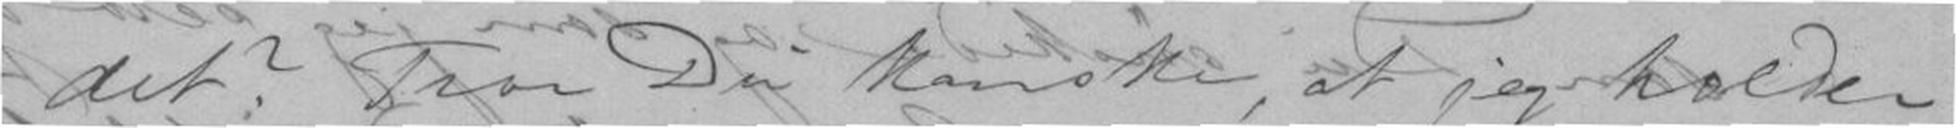det? Tror Du kanske, at jeg holder
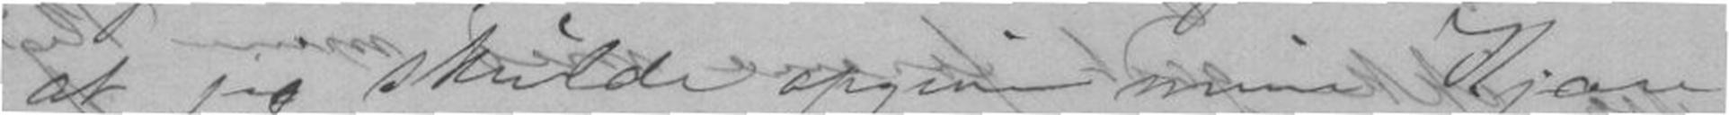at jeg skulde opgive mine Kjære,
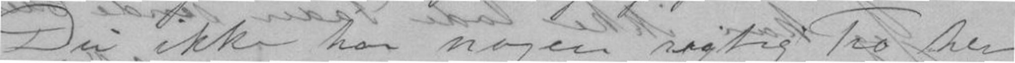Du ikke har nogen rigtig Tro her-
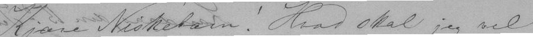Kjære Niskebarn! Hvad skal jeg vel
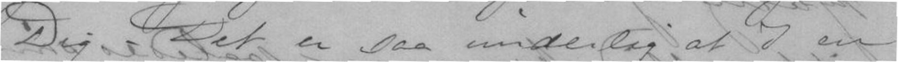Dig, Det er saa underlig at I ere
